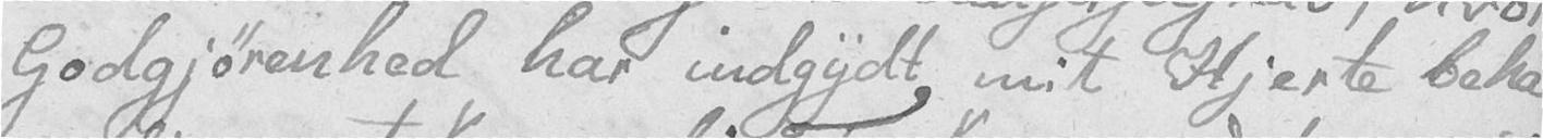Godgjørenhed har indgydt mit Hjerte beha
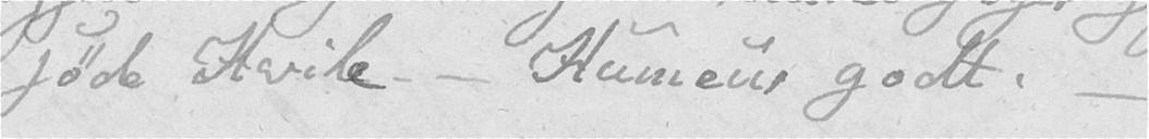søde Hvile. – Humeur godt. –
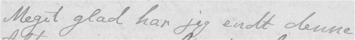Meget glad har jeg endt denne
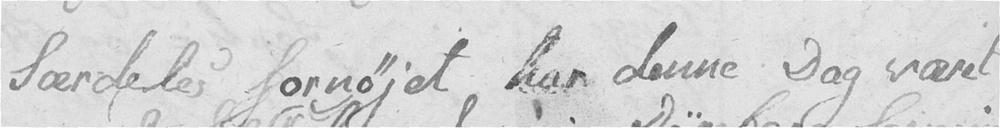Særdeles fornøjet har denne Dag været
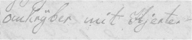omkryber mit Hjerte. –
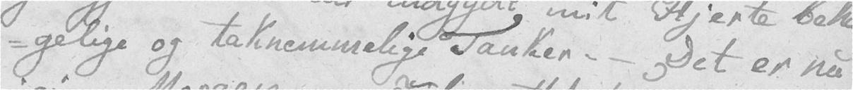-gelige og taknemmelige Tanker. – Det er nu
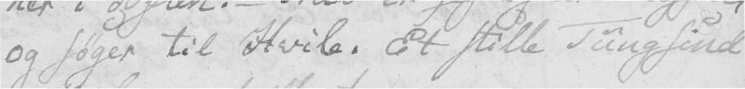og søger til Hvile. Et stille Tungsind
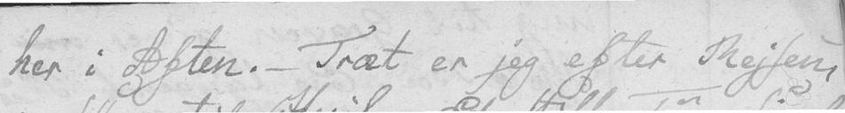her i Aften. – Træt er jeg efter Rejsen,
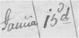Janua 15d
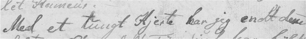Med et tungt Hjerte har jeg endt denne
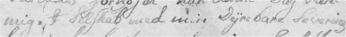mig. I Selskab med min Dyrebare Severine
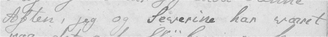Aften, jeg og Severine har været
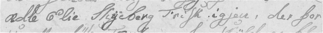ædle Elie Skyeberg Frisk igjen, der for
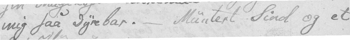mig saa Dyrebar. – Muntert Sind og et
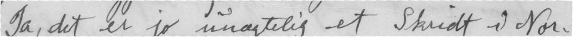Ja, det er jo unægtelig et Skridt i Nor-
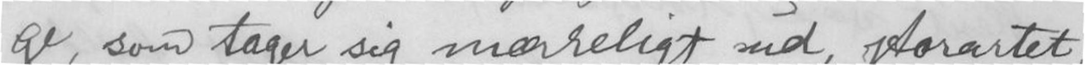ge, som tager sig mærkeligt ud, storartet,
